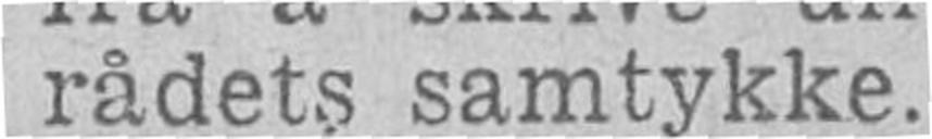rådets samtykke.
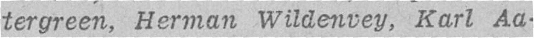tergreen, Herman Wildenvey, Karl Aa-
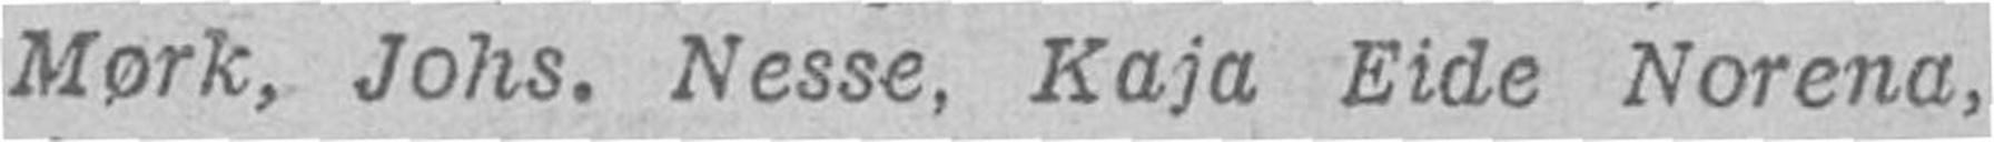Mørk, Johs. Nesse, Kaja Eide Norena,
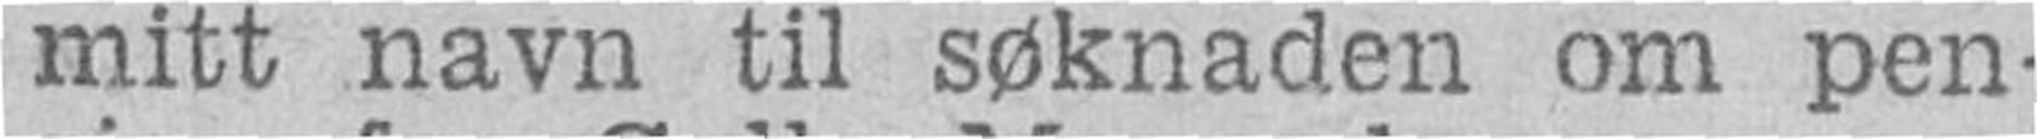mitt navn til søknaden om pen-
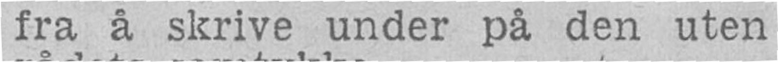fra å skrive under på den uten
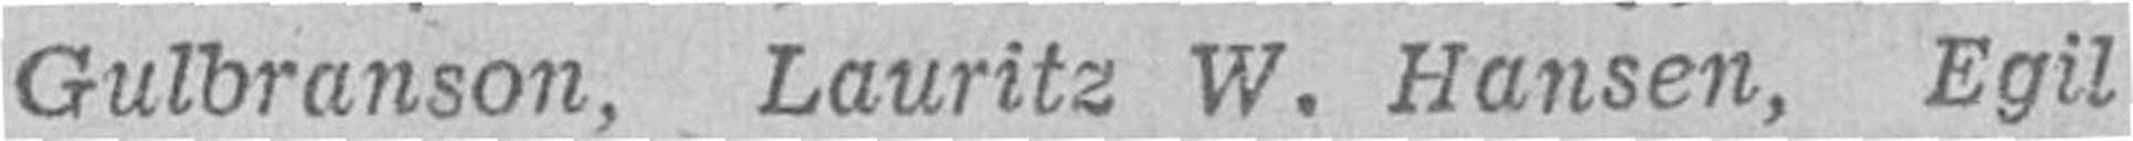Gulbranson, Lauritz W. Hansen, Egil
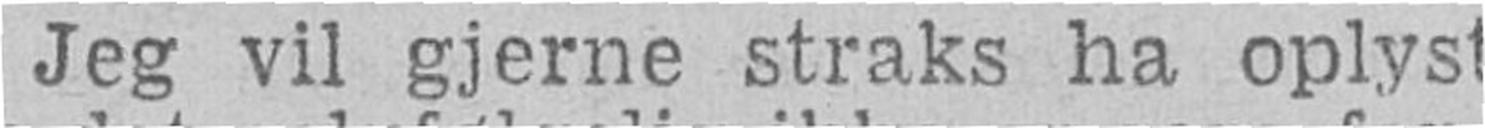Jeg vil gjerne straks ha oplyst
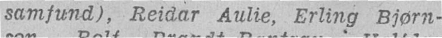samfund), Reidar Aulie, Erling Bjørn-
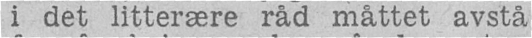i det litterære råd måttet avstå
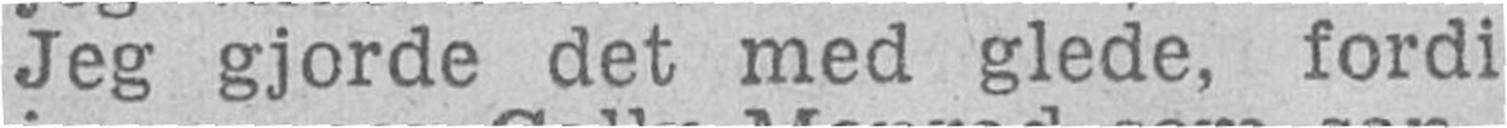Jeg gjorde det med glede, fordi
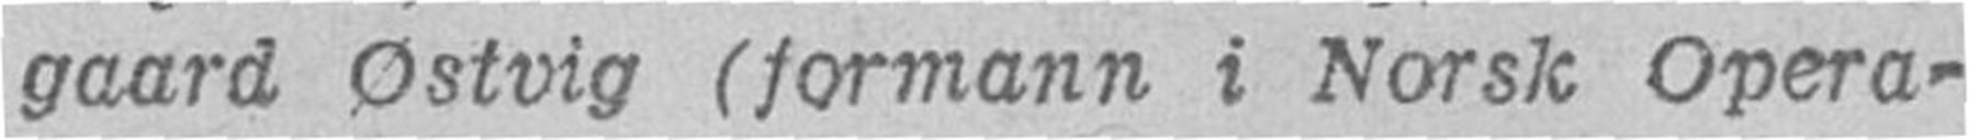gaard Østvig (formann i Norsk Opera-
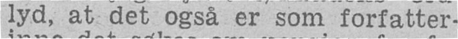lyd, at det også er som forfatter-
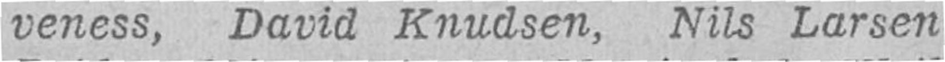veness, David Knudsen, Nils Larsen
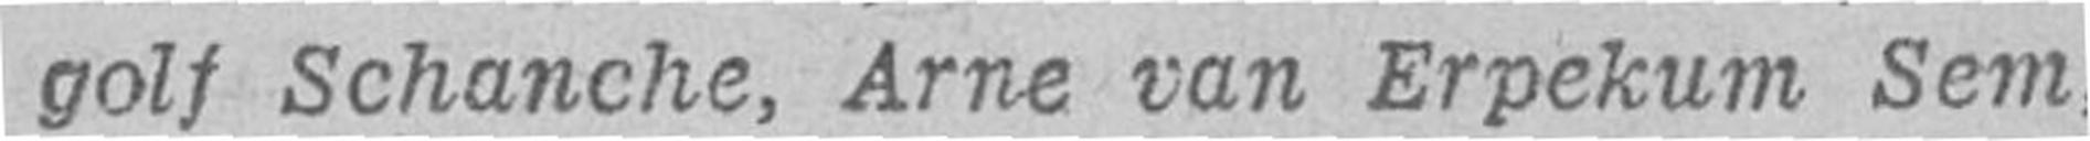golf Schanche, Arne van Erpekum Sem,
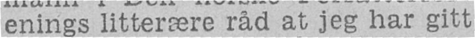enings litterære råd at jeg har gitt
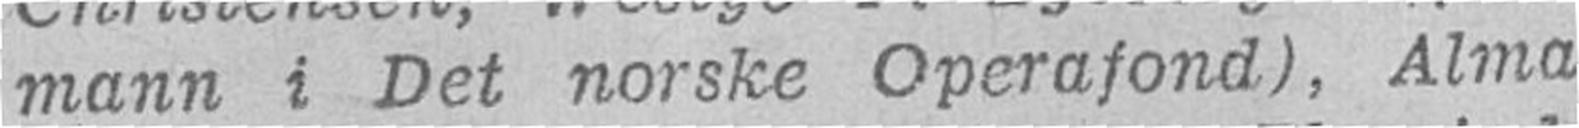mann i Det norske Operafond), Alma
